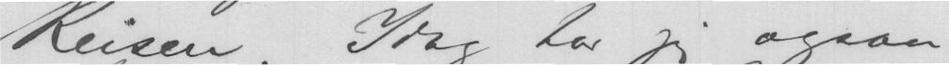Reisen. Idag har jeg ogsaa
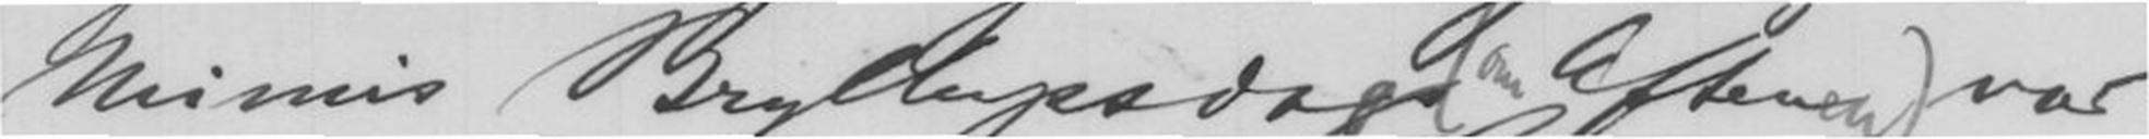Mimis Bryllupsdag (om Aftenen) var
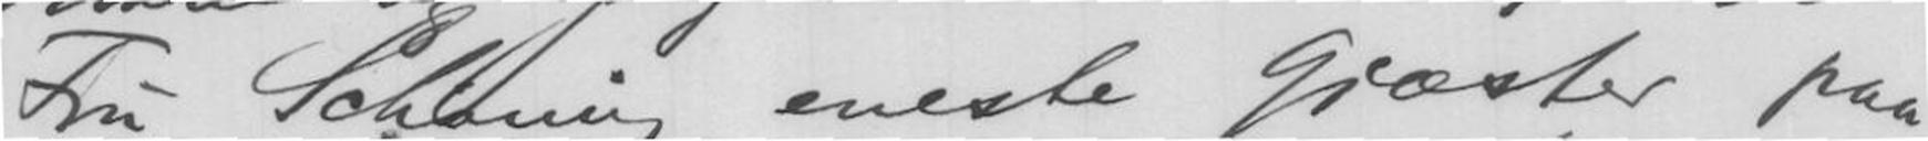Fru Schøning eneste Gjæster paa
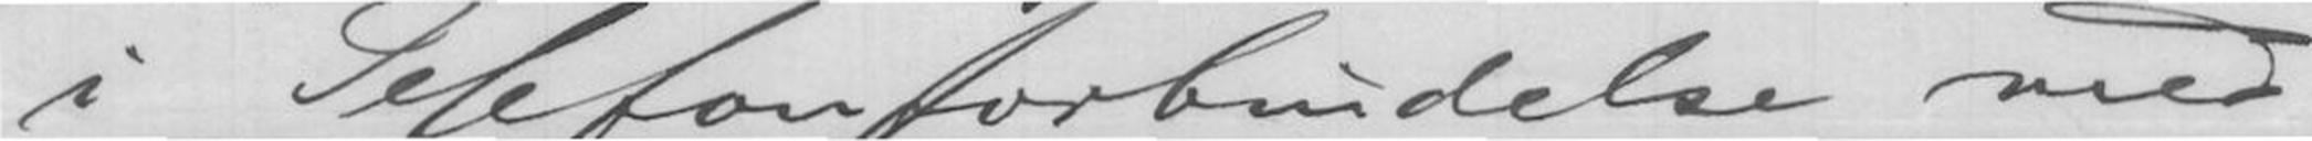i Telefonforbindelse med
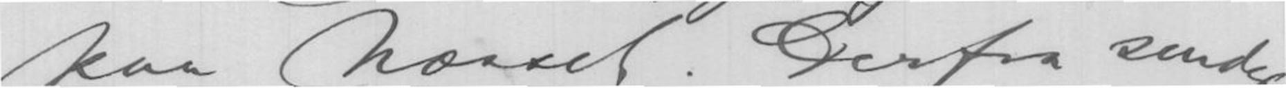paa Næsset. Derfra sender
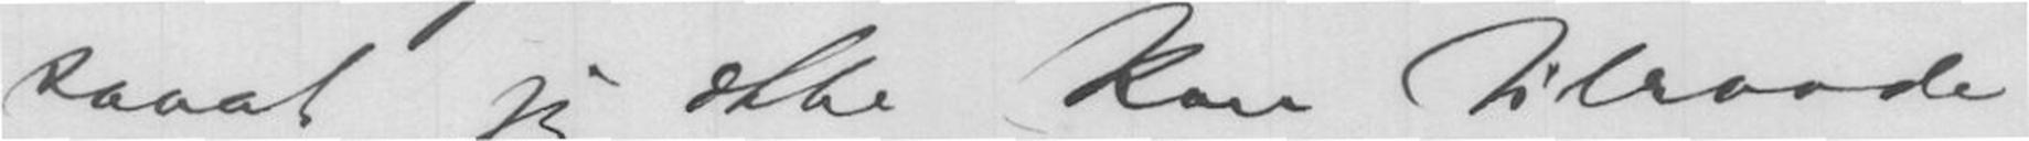saaat jeg ikke kan tilraade
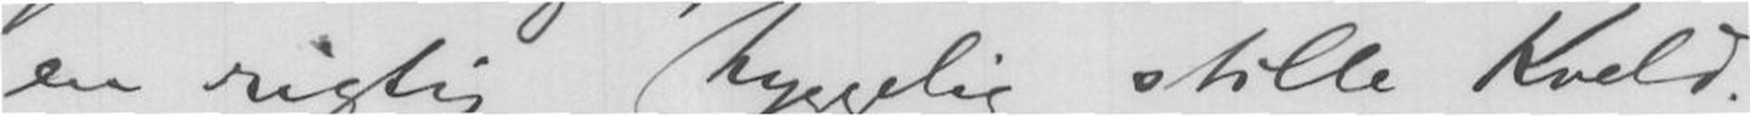en rigtig hyggelig stille Kveld.
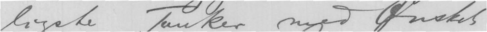ligste Tanker med Ønsket
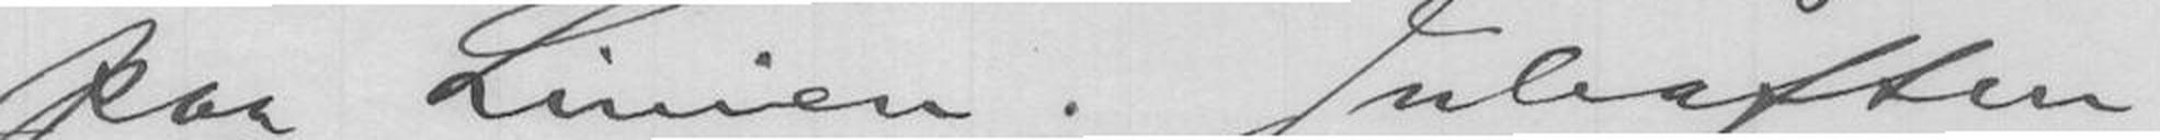paa Linien. Julaften
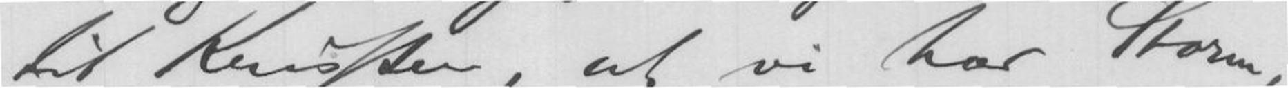til Kristen, at vi har Storm,
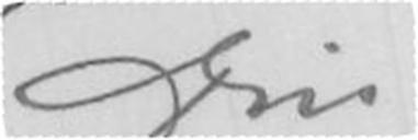Din
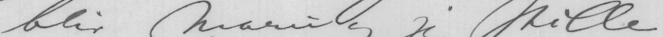blir Marie og jeg stille
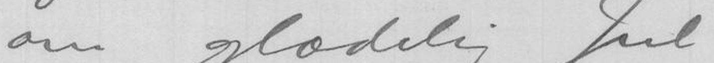om glædelig Jul!
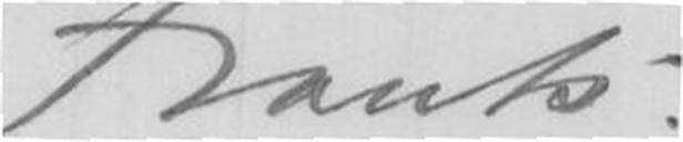Frants.
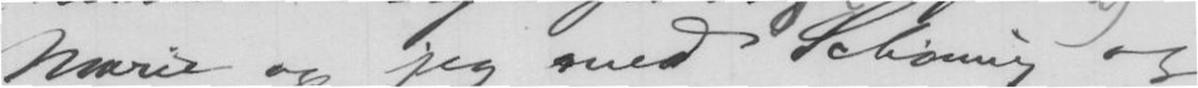Marie og jeg med Schøning og
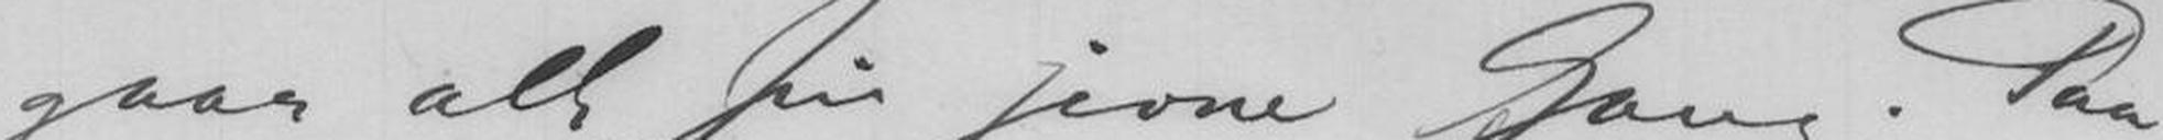gaar alt sin jevne Gang. Paa
